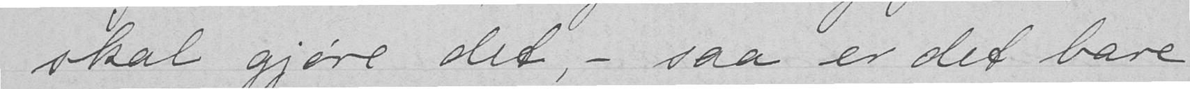skal gjøre det, - saa er det bare
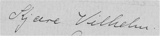Kjære Vilhelm!
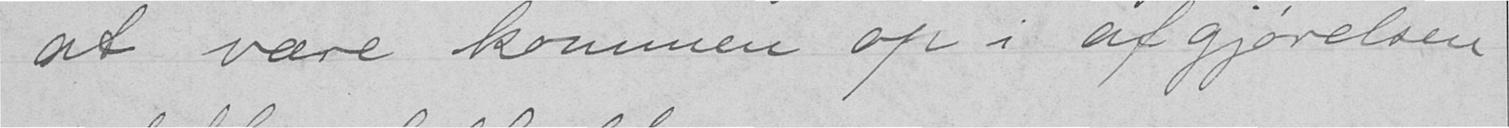at være kommen op i afgjørelsen
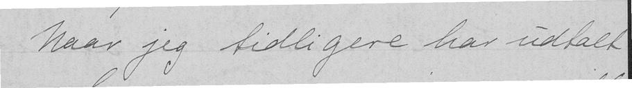Naar jeg tidligere har udtalt
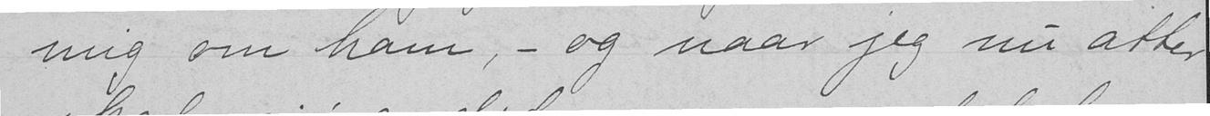mig om ham, - og naar jeg nu atter
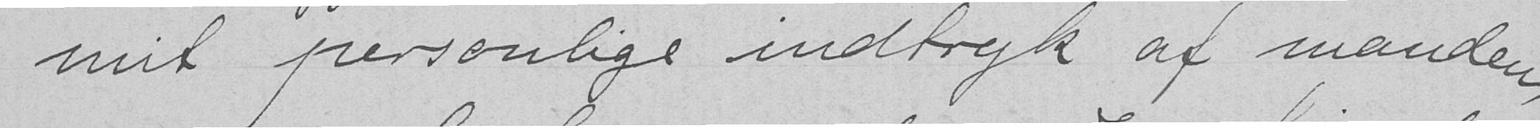mit personlige indtryk af manden,
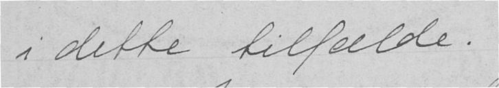i dette tilfælde.
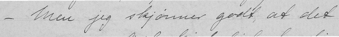- Men jeg skjønner godt, at det
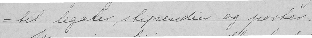- til legater, stipendier og poster.
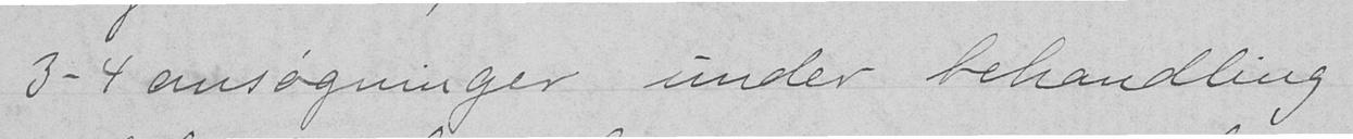3-4 ansøgninger under behandling
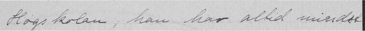Høgskolan; han har altid mindst
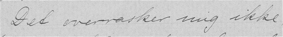Det overrasker mig ikke,
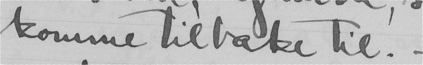komme tilbake til. -
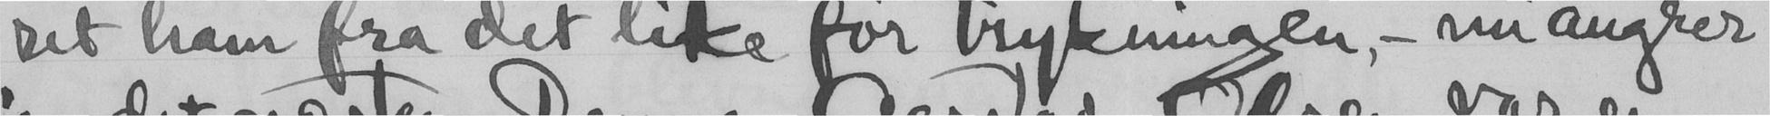ret ham fra det like før trykningen, - nu angrer
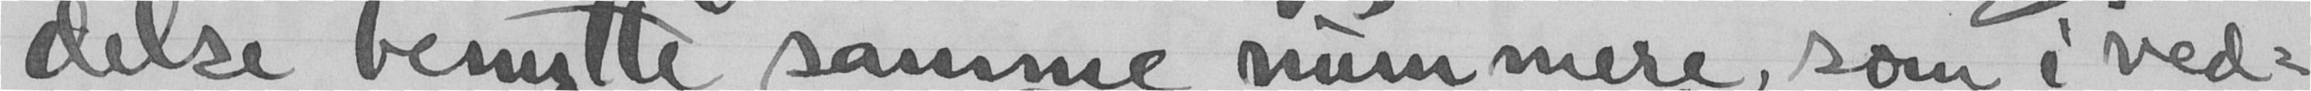delse benytte samme nummere, som i ved-
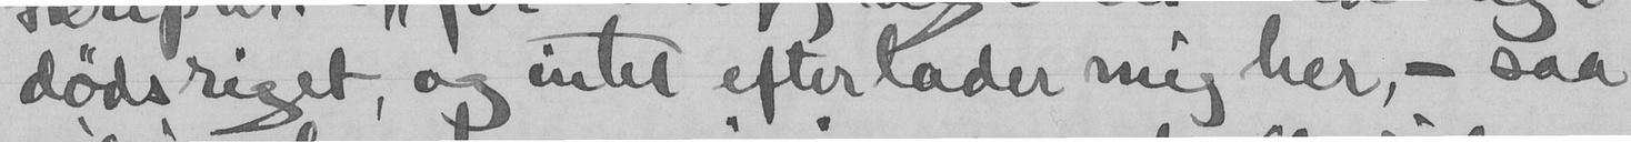dødsriget og intet efterlader mig her, - saa
